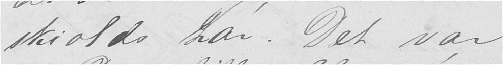skiolds har. Det var
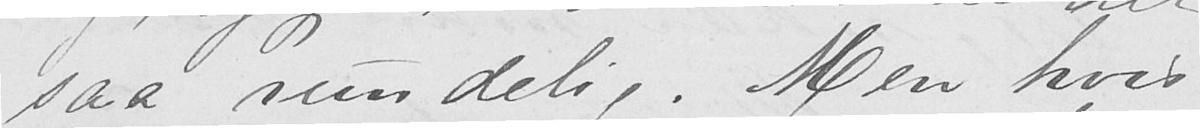saa rundelig. Men hvis
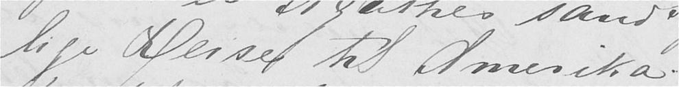lige Reise til Amerika.
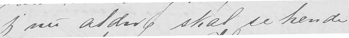jeg nu aldrig skal se hende
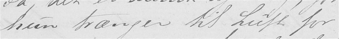hun trænger til Luft for
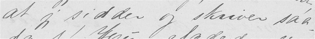at jeg sidder og skriver saa-
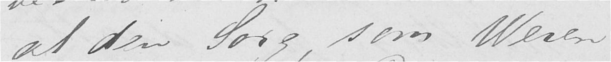af den Sorg, som Weren-
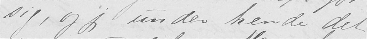sig, og jeg under hende det
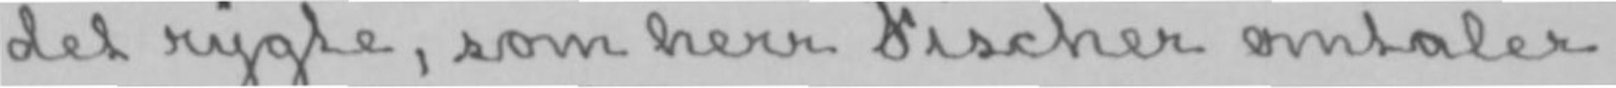det rygte, som herr Fischer omtaler
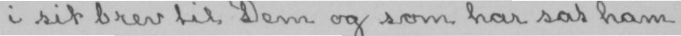i sit brev til Dem og som har sat ham
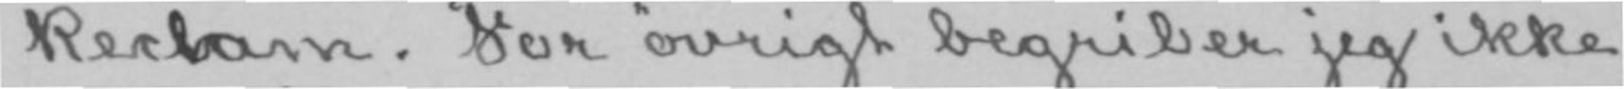Reclam. For øvrigt begriber jeg ikke
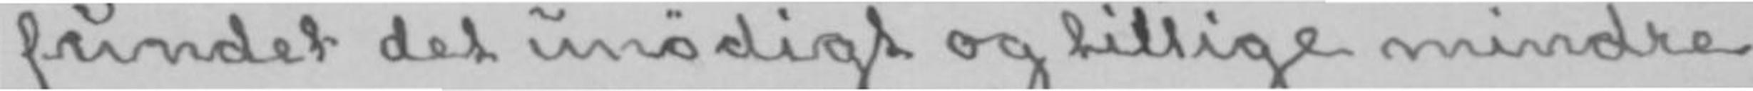fundet det unødigt og tillige mindre
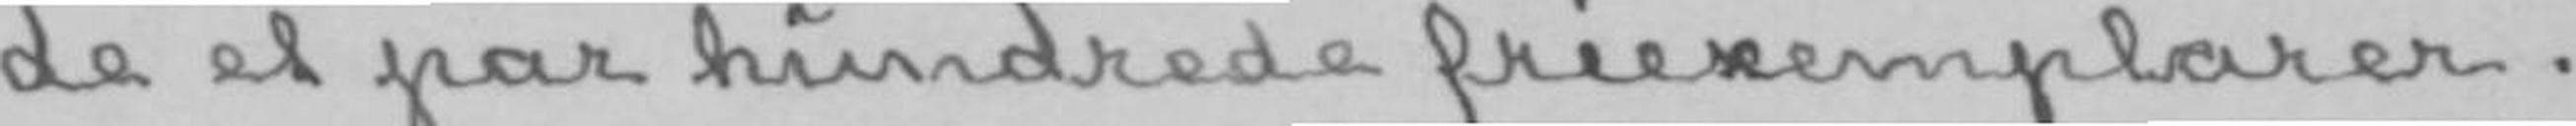de et par hundrede friexemplarer.
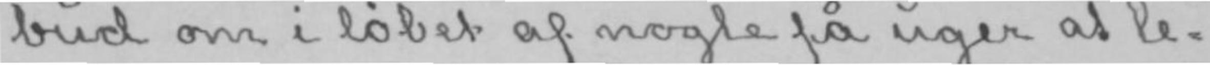bud om i løbet af nogle få uger at le-
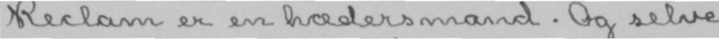Reclam er en hædersmand. Og selve
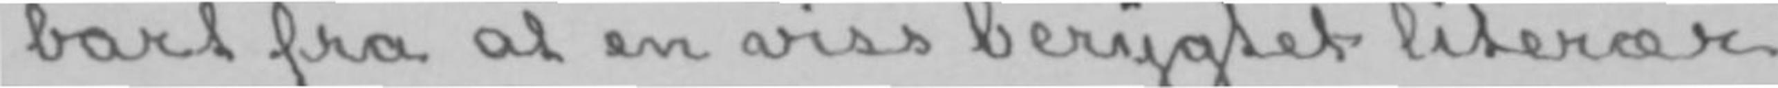bart fra at en viss berygtet literær
